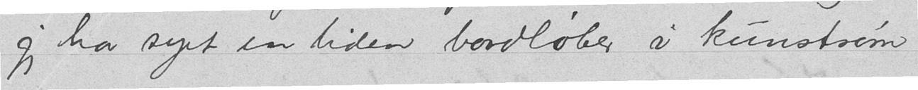jeg har syet en liden bordløber i kunstsøm
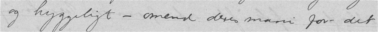og hyggeligt – omend deres mani for det
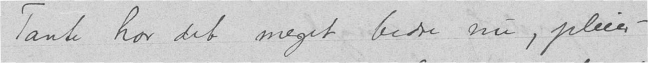Tante har det meget bedre nu, pleier-
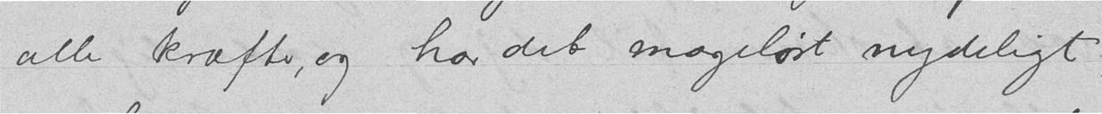alle kræfter, og har det mageløst nydeligt
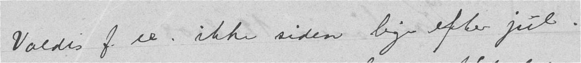Valdis f.ex. ikke siden lige efter jul.
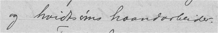og hvidtsøms haandarbeider.
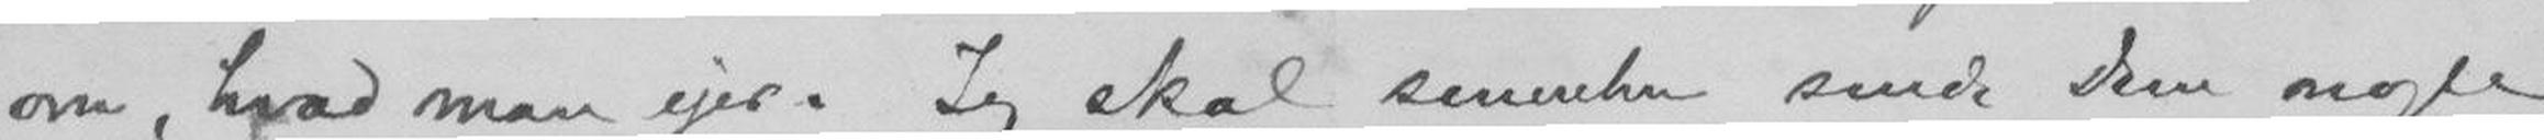om, hvad man ejer. Jeg skal senerehen sende Dem nogle
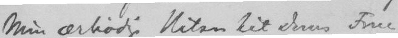Min ærbødige Hilsen til Deres Frue
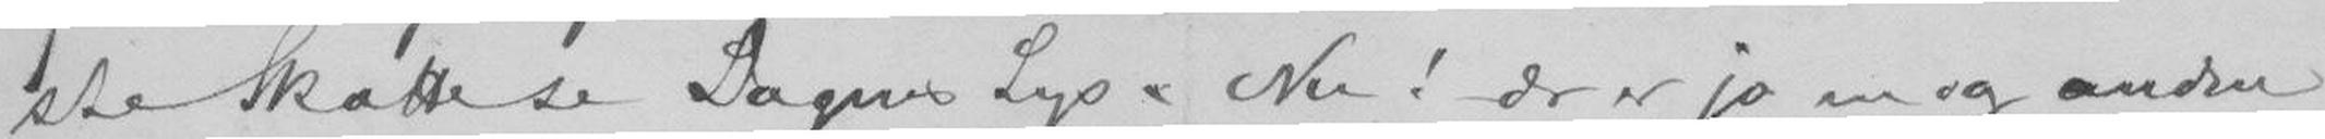ste Skatte se Dagens Lys. Nu! der er jo en og anden
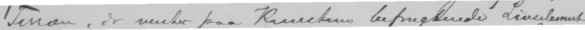Terræn, der venter pa kunstens befrugtende Livselement,
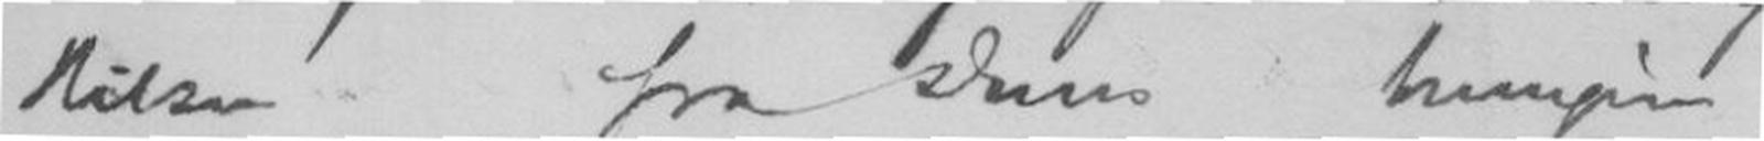Hilsenfra Deres hengivne
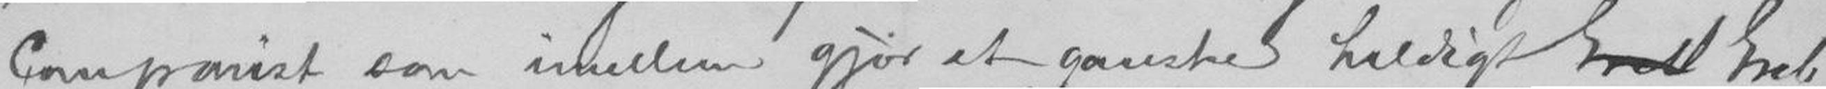Componist som imellem gjør et ganske heldigt Greb
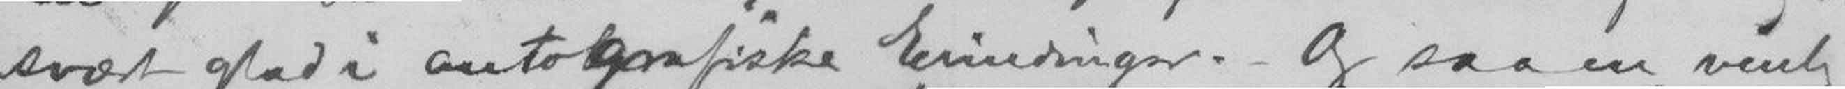svært glad i autografiske Erindinger. - Og saa en venlig
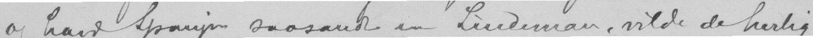og havd Spanjen saasant en Lindeman, vilde de herlig
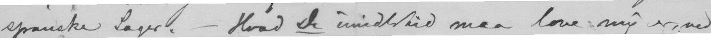spanske Sager. Hvad De imidlertid maa love mig er, ved
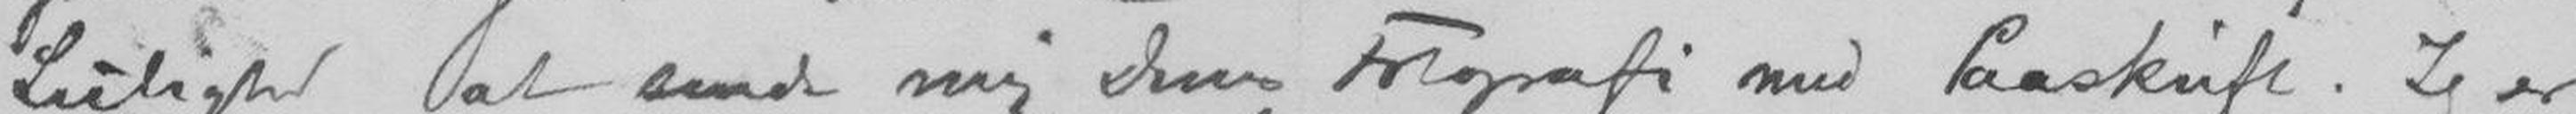Leilighed at sende mig Deres Fotografi med Paaskrift. Jeg er
